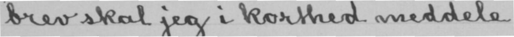brev skal jeg i korthed meddele
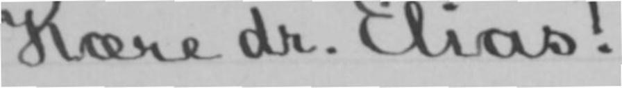Kære dr. Elias!
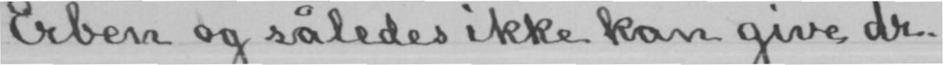Erben og således ikke kan give dr.
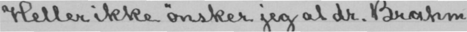Heller ikke ønsker jeg at dr. Brahm
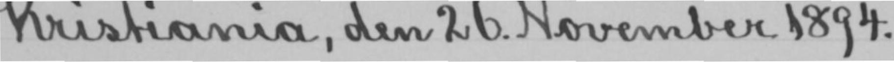Kristiania, den 26. November 1894.
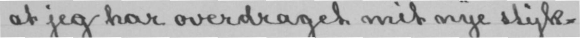at jeg har overdraget mit nye styk-
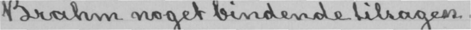Brahm noget bindende tilsage.n.
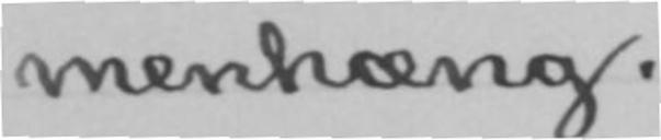menhæng.
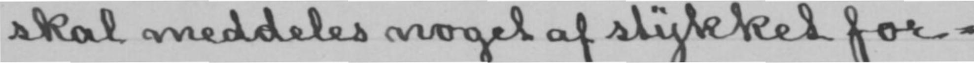skal meddeles noget af stykket for-
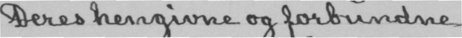Deres hengivne og forbundne
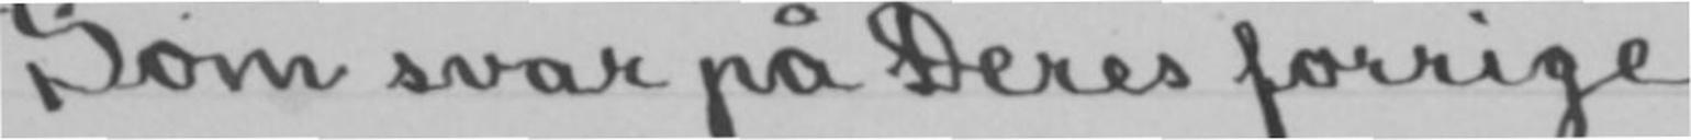Som svar på Deres forrige
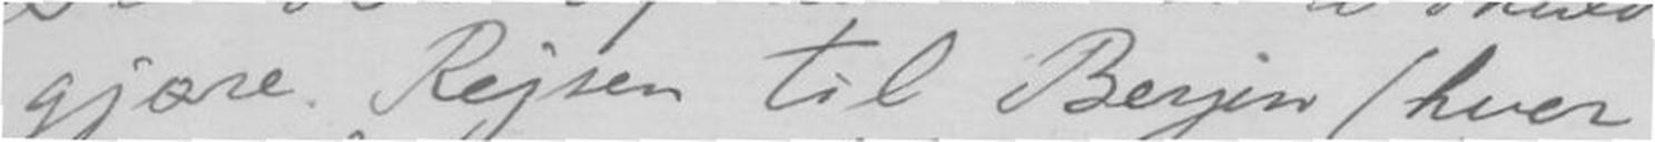gjøre Rejsen til Berjen (hvor
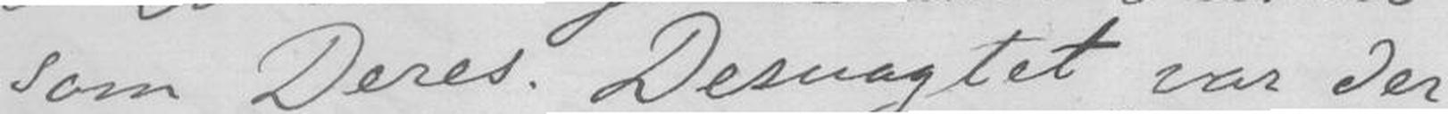som Deres. Desuagtet var der
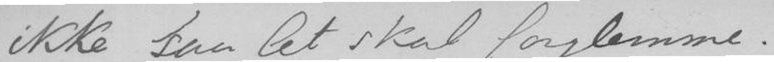ikke saa let skal forglemme.
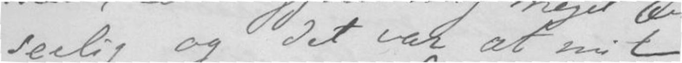seelig og det var at mit
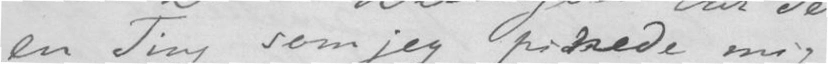en Ting som jeg pakede mig
